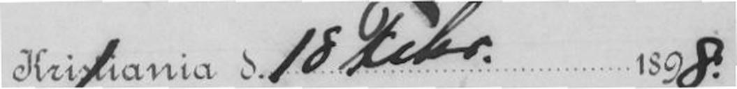Kristiania d. 18 Febr. 1898.
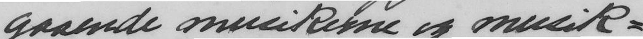gaaende musikerne og musik-
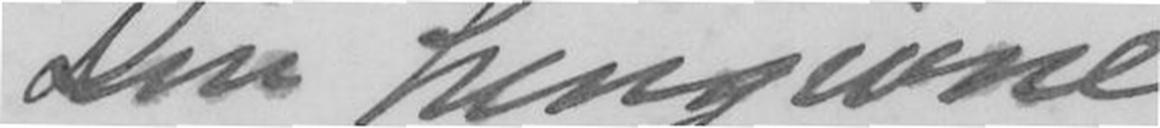Din hengivne
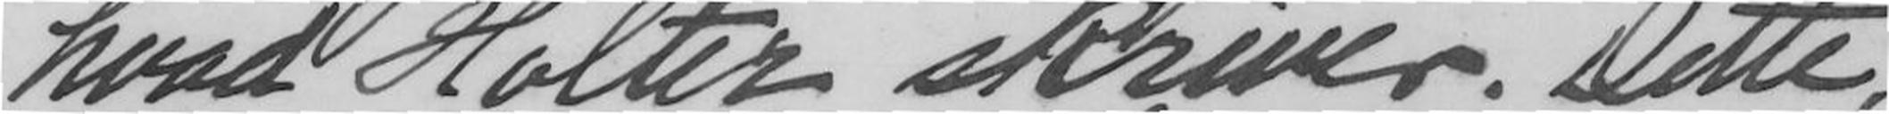hvad Holter skriver. Dette,
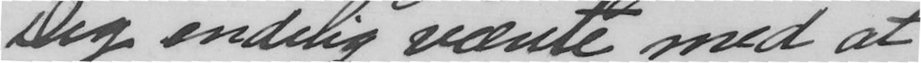Dig endelig vænte med at
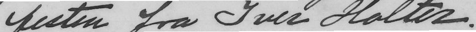festen fra Iver Holter. -
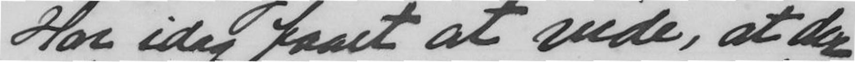Har idag faaet at vide, at der
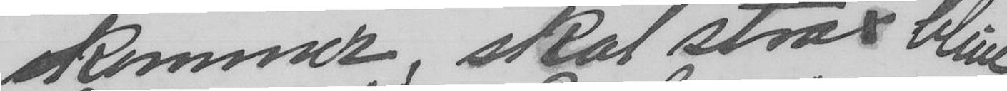kommer, skal strax blive
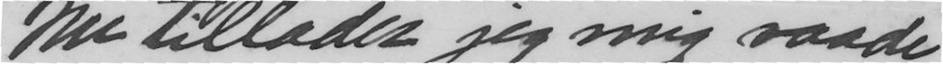Nu tillader jeg mig raade
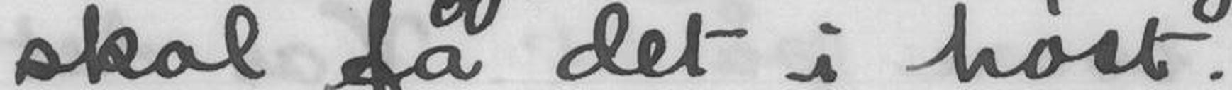skal få det i høst.
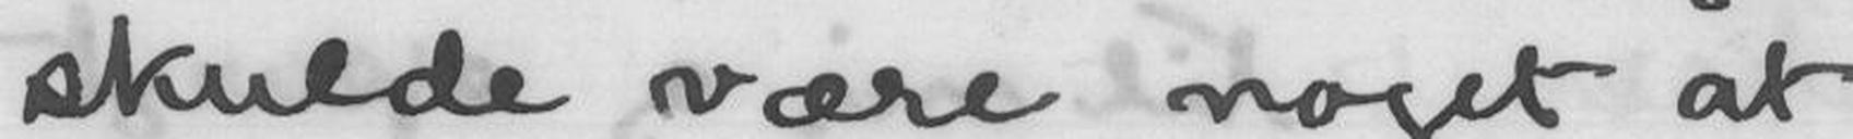skulde være noget at
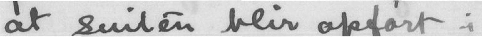at suiten blir opført i
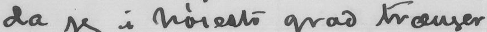da jeg i høieste grad trænger
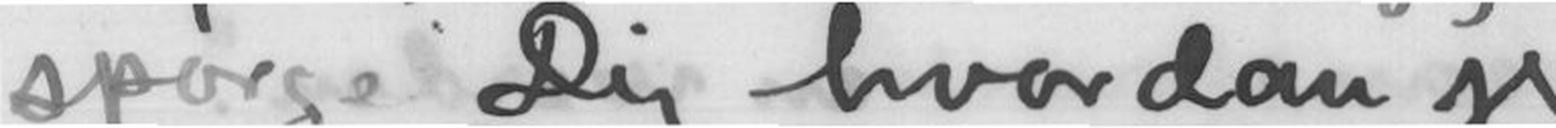spørge Dig hvordan jeg
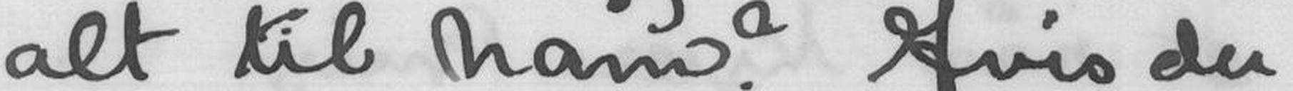alt til ham? Hvis du
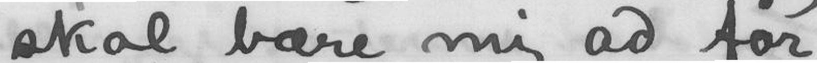skal bære mig ad for
